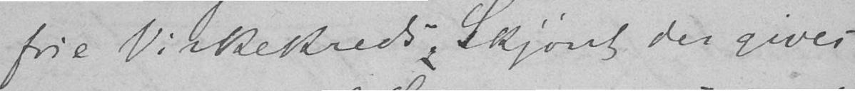frie Virkekreds. Skjønt der gives
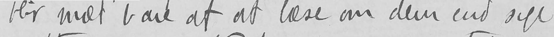blir mæt bare af at læse om dem end sige
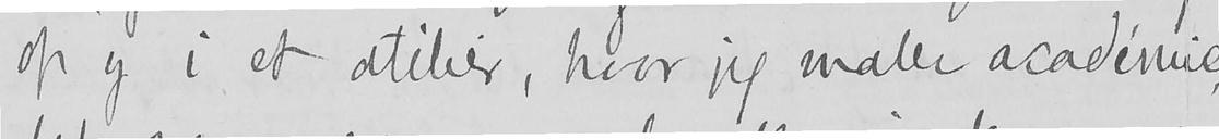op og i et atélier, hvor jeg maler académie,
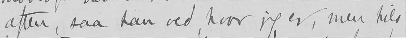aften, saa han ved, hvor jeg er, men hils
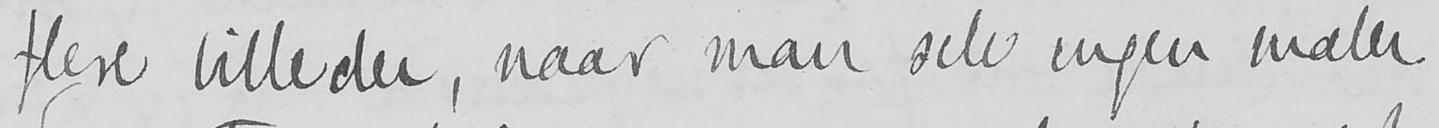flere billeder, naar man selv ingen maler
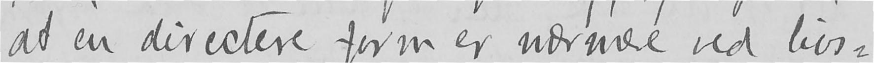at en directere form er nærmere ved livs-
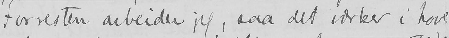Forresten arbeider jeg, saa det værker i hove-
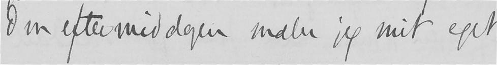Om eftermiddagen maler jeg mit eget
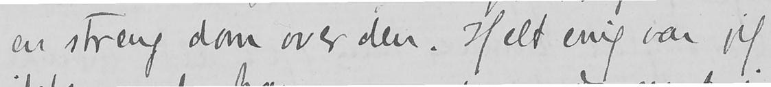en streng dom over den. Helt enig var jeg
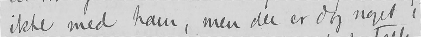ikke med ham, men der er dog noget i
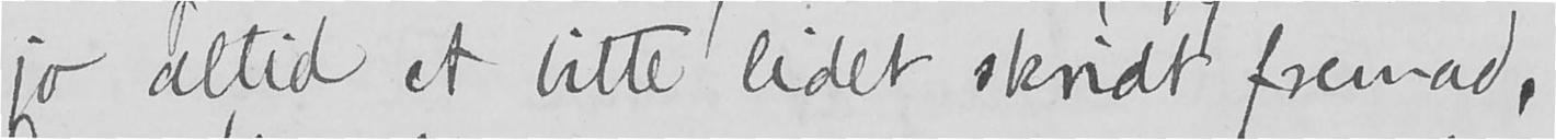jo altid et bitte lidet skridt fremad.
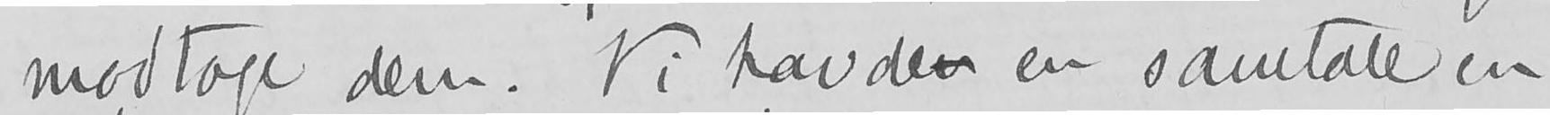modtage dem. Vi havden en samtale en
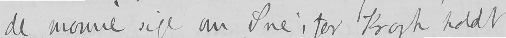de monne sige om "Sne", for Krogh holdt
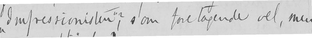"Impressionisten", som foretagende vel, men
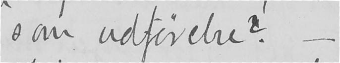som udførelse? -
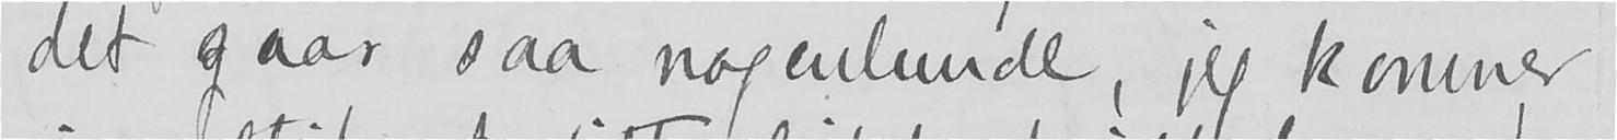det gaar saa nogenlunde, jeg kommer
